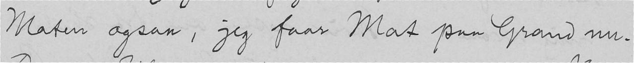Maten ogsaa, jeg faar Mat paa Grand nu.
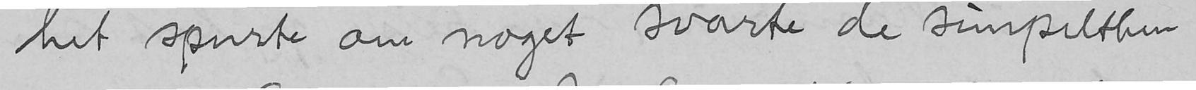het spurte om noget svarte de simpelthen
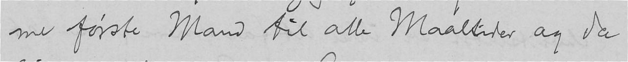me første Mand til alle Maaltider og da
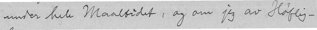under hele Maaltidet, og om jeg av Høflig-
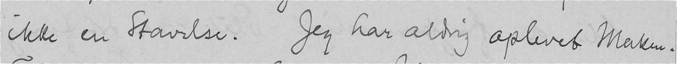ikke en Stavelse. Jeg har aldrig oplevet Maken.
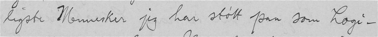ligste Mennesker jeg har støtt paa som Logi-
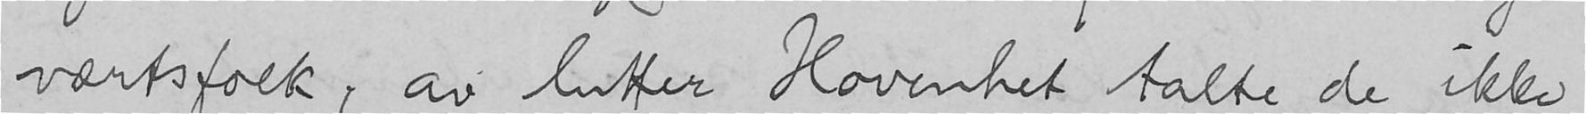værtsfolk, av lutter Hovenhet talte de ikke
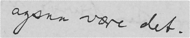ogsaa være det.
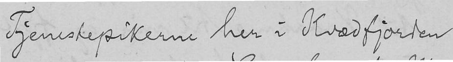Tjenestepikerne her i Kvædfjorden
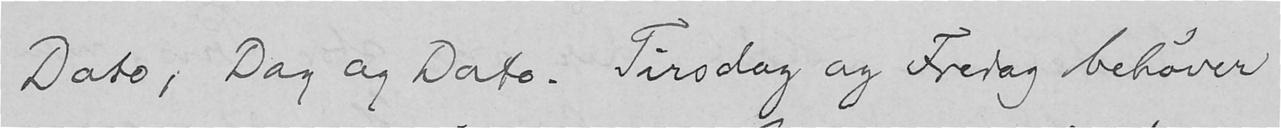Dato, Dag og Dato. Tirsdag og Fredag behøver
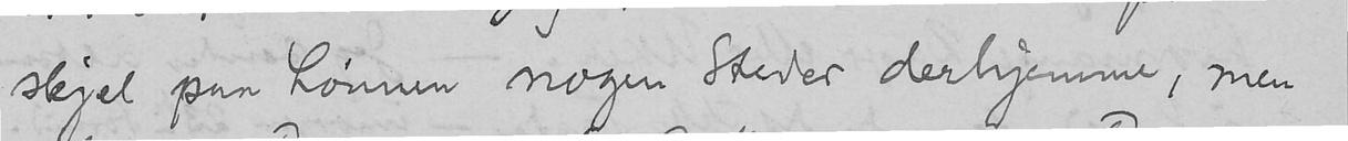skjel paa Lønnen nogen Steder derhjemme, men
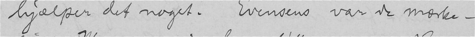hjælper det noget. Evensens var de mærke-
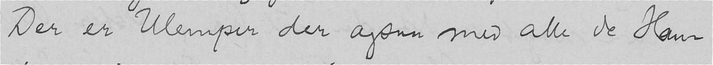Der er Ulemper der ogsaa med alle de Han-
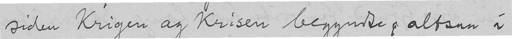siden Krigen og Krisen begyndte, altsaa i
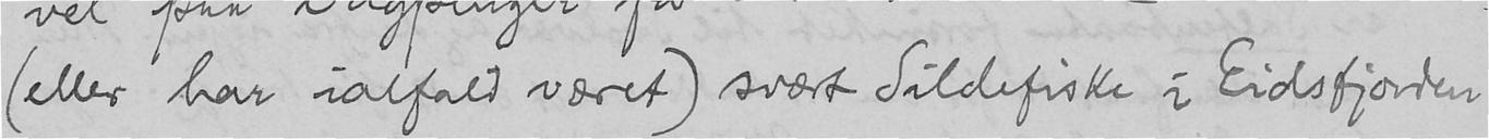(eller har ialfald været) svært Sildefiske i Eidsfjorden
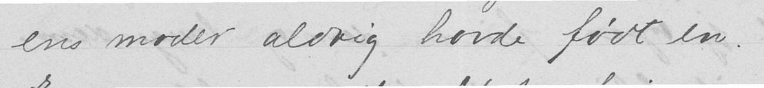ens moder aldrig havde født en.
